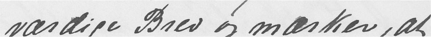værdige Brev og mærker, at
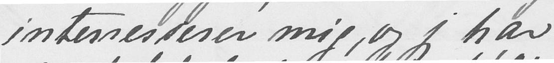interesserer mig, og jeg har
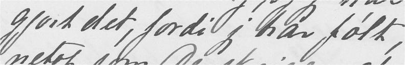gjort det, fordi jeg har følt,
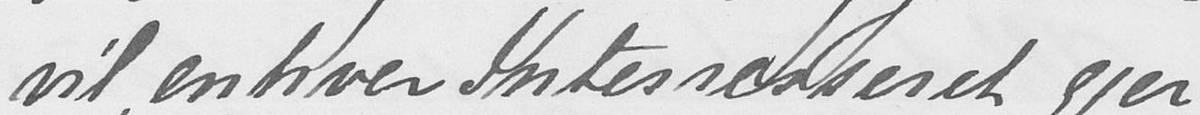vil "enhver Interreseret gjer-
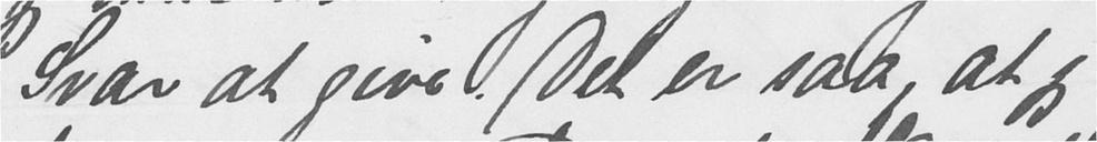Svar at give. Det er saa, at jeg
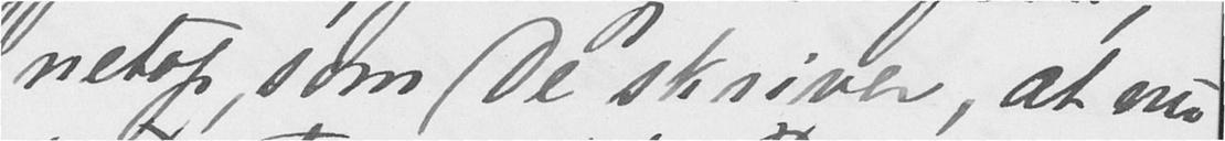netop, som De skriver, at nu
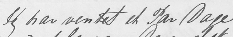Jeg har ventet et Par Dage
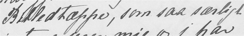Billedtæppe, som saa særligt
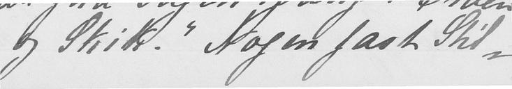og Skik." Nogen fast Stil-
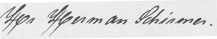Hr Herman Schirmer.
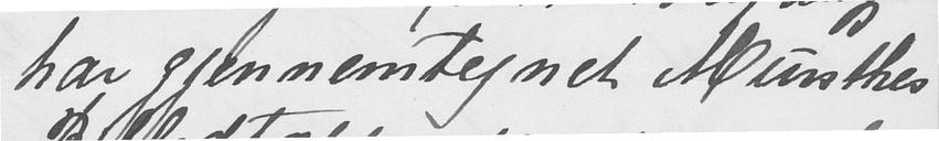har gjennemtegnet Munthes
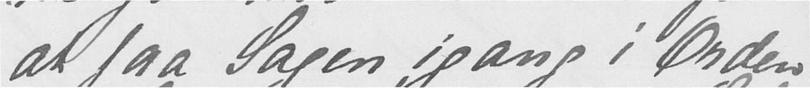at faa Sagen igang i Orden
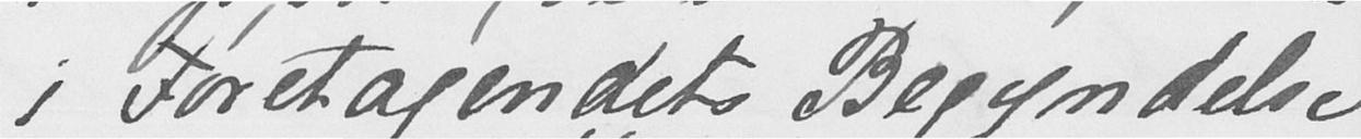i Foretagendets Begyndelse
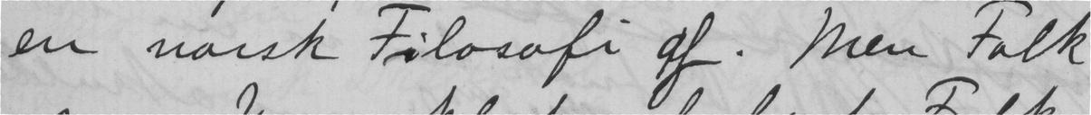en norsk Filosofi af. Men Folk
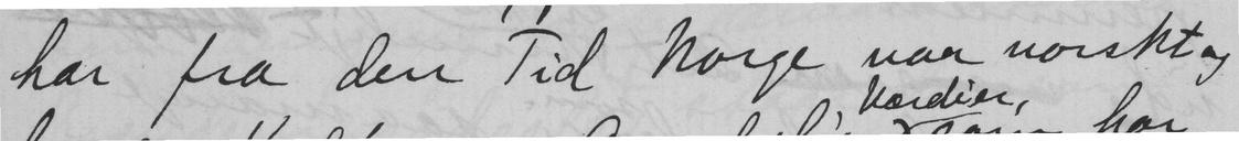har fra den Tid Norge var norskt og
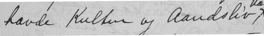havde Kultur og Aandsliv,
